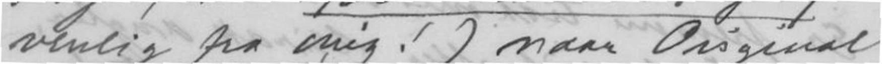venlig fra mig!) naar Original
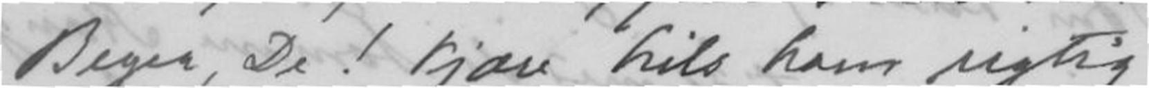Beyer, De! kjære hils ham rigtig
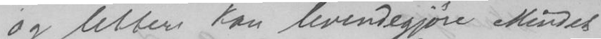og lettere kan levendegjøre Mindet
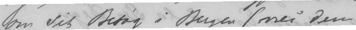om sit Besøg i Bergen (nei den
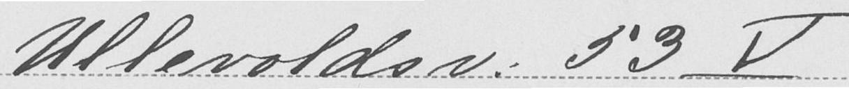Ullevoldsv. 53 V
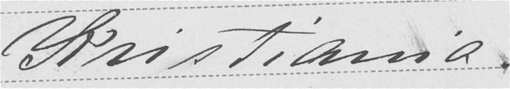Kristiania.
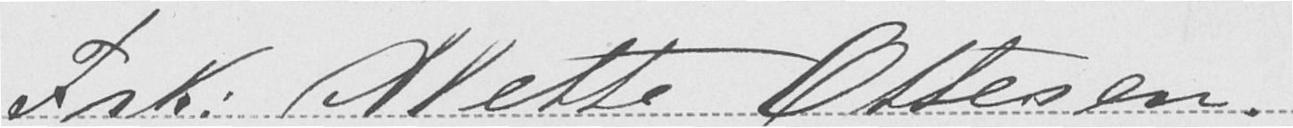Frk. Alette Ottesen.
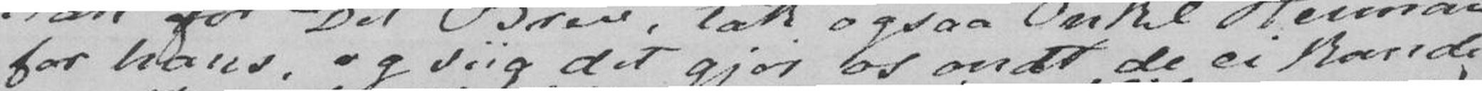for hans, og siig det gjør os ondt de ei kunde
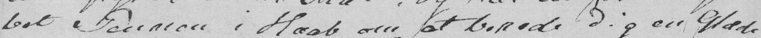bet Pennen i Haab om at berede Dig en Glæde
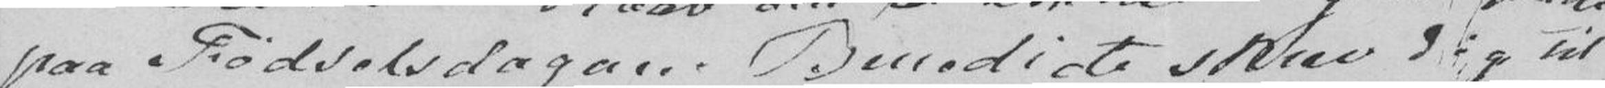paa Fødselsdagen. Benedicte skrev Dig til
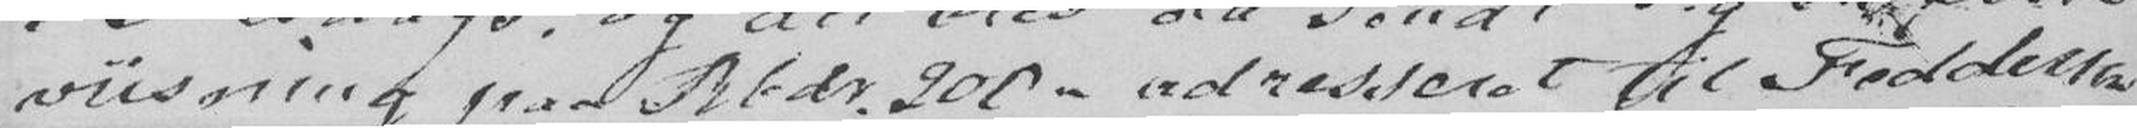viisning paa Rbdr. 200- adresseret til Feddersen
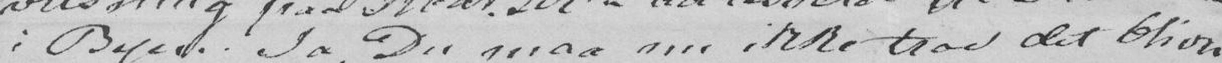i Byen. Ja Du maa nu ikke troe det bliver
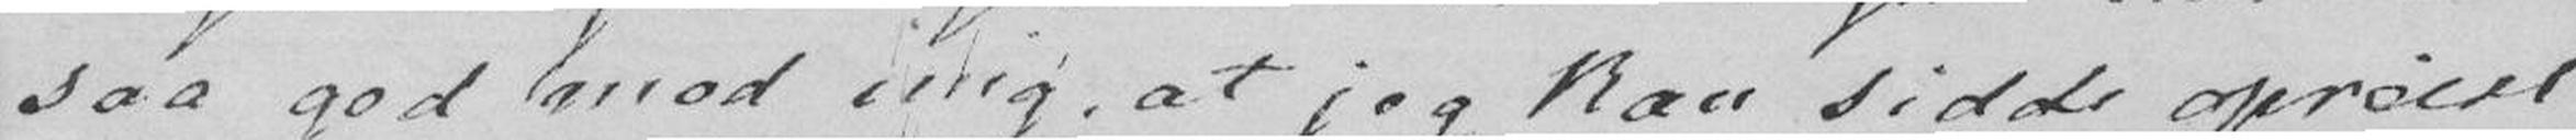saa god mod mig, at jeg kan sidde opreist
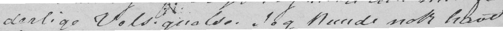derlige Velsignelse. Jeg kunde nok havt
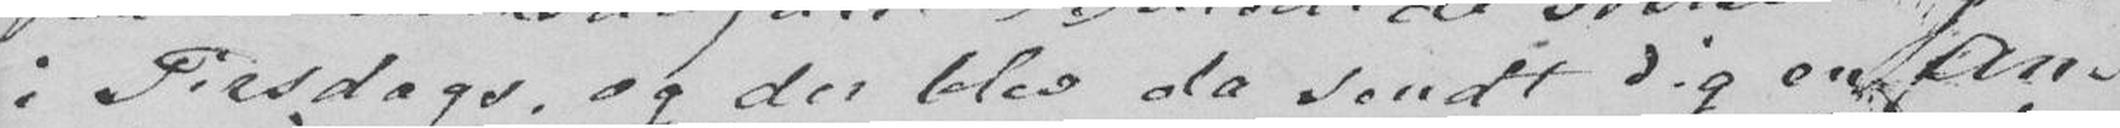i Tirsdag, og der blev da sendt Dig en An-
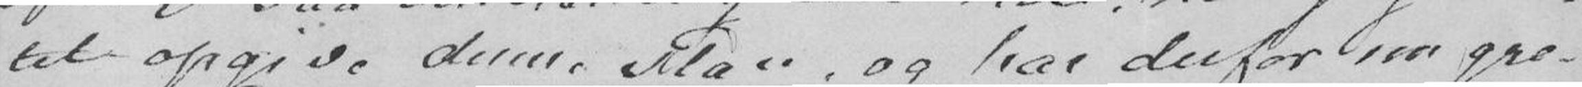tet opgive denne Plan, og har derfor nu gre-
